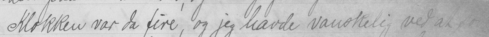Klokken var da fire, og jeg havde vanskelig ved at sove
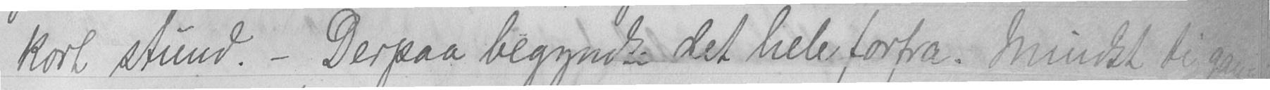kort stund. - Derpaa begyndte det hele forfra. Mindst ti gan-
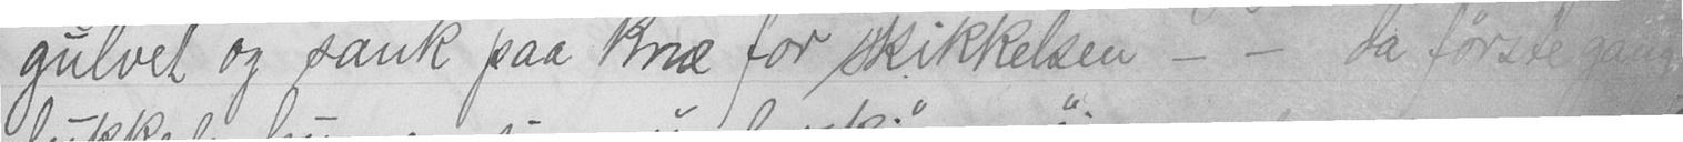guevet og sank paa knæ for skikkelsen - - da første gang
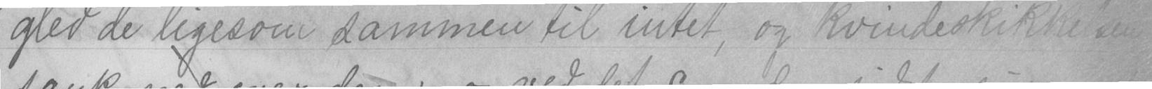gled de ligesom sammen til intet, og kvindeskikkelsen
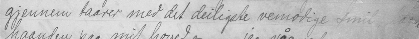gjennem taarer med det deiligste vemodige smil, lagede
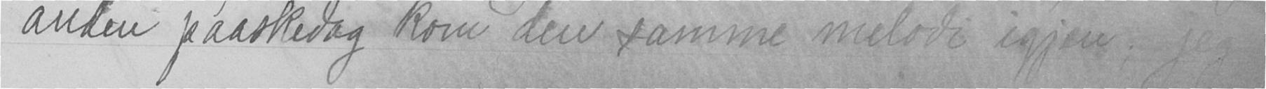anden paaskedag kom den samme melodi igjen; jeg
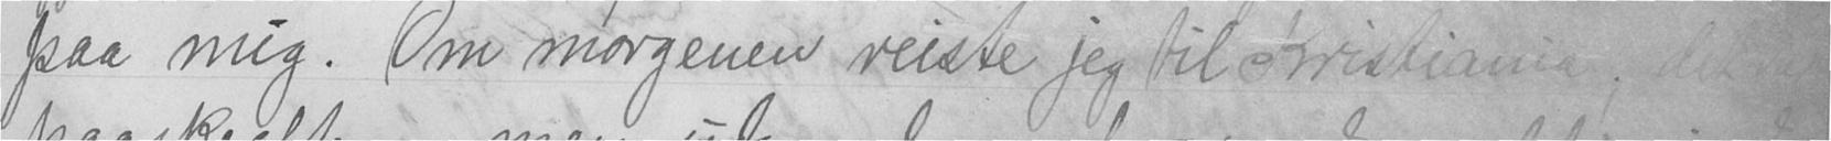paa mig. Om morgenen reiste jeg til Kristiania; det var
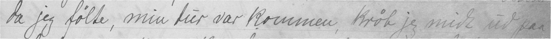da jeg følte, min tur var kommen, krøb jeg midt ud paa
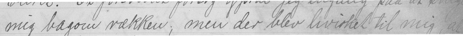mig bagom rækken; men der blev hvisket til mig, at
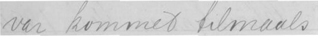var kommet tilmaals.
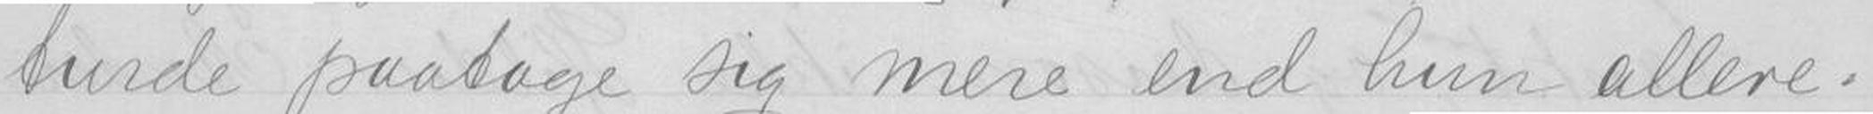turde paatage sig mere end hun allere-
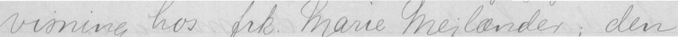visning hos frk. Marie Mejlænder; den
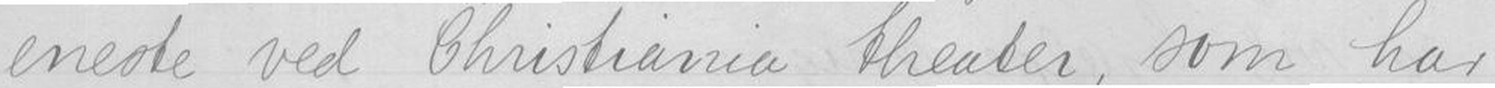eneste ved Christiania theater, som har
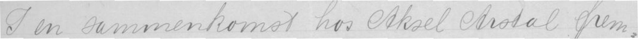I en sammenkomst hos Aksel Arstal frem-
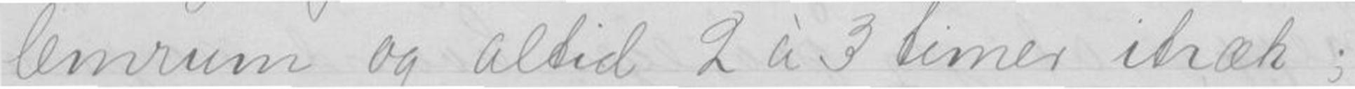lemrum og altid 2 à 3 timer itræk;
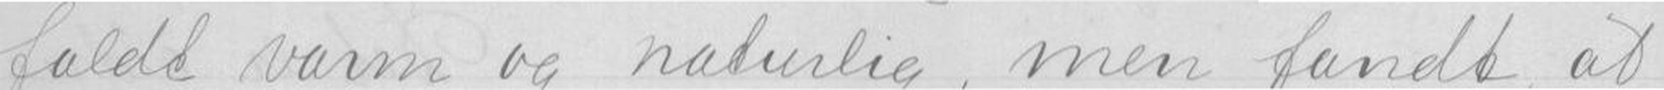faldt varm og naturlig, men fandt, at
